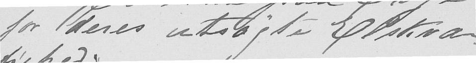for deres utsøgte Elskvæ-
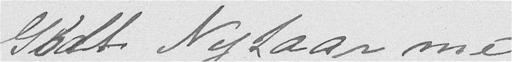Gldt Nytaar med
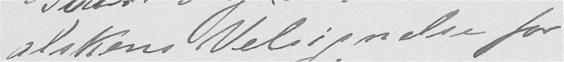alskens Velsignelse for
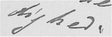dighed.
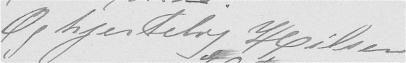Og hjertelig Hilsen
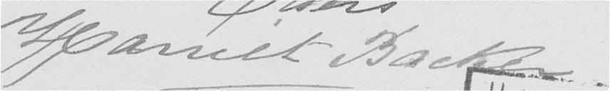Harriet Backer.
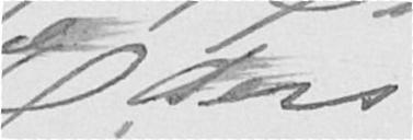Eders
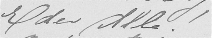Eder Alle!
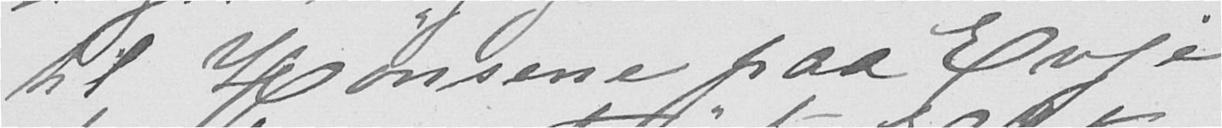til Hønsene paa Evje
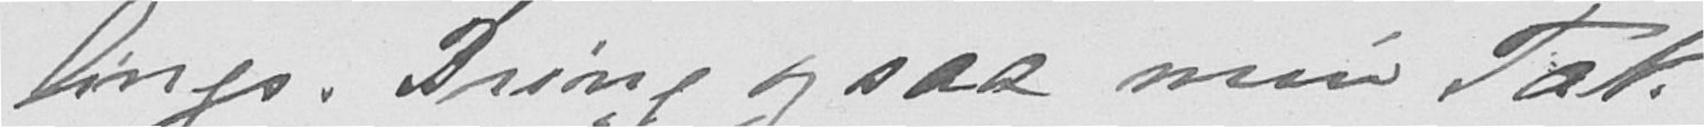lings. Bring ogsaa min Tak
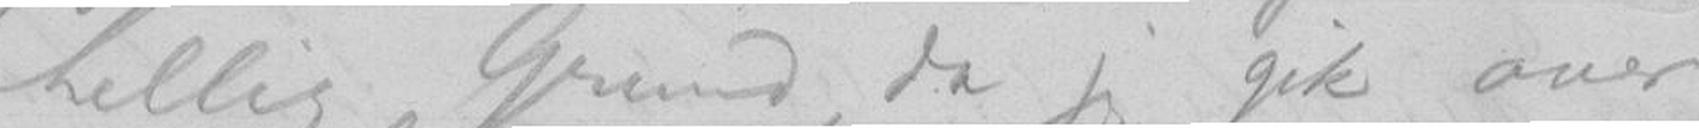hellig Grund, da jeg gik over
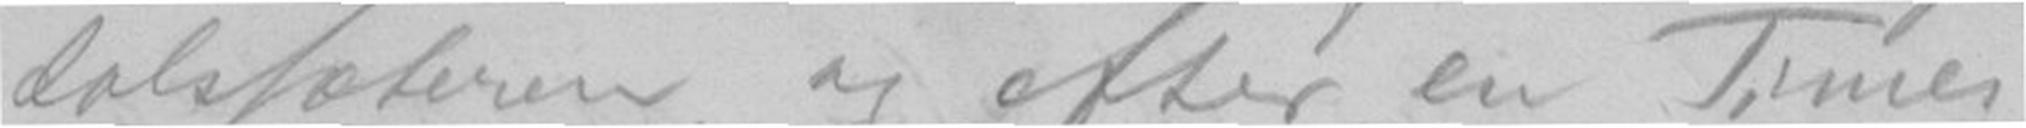dalssæteren og efter en Times
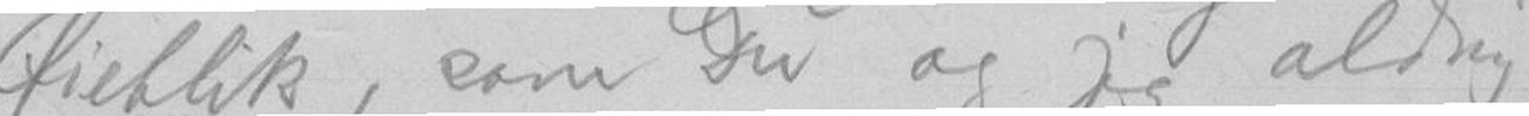Øieblik, som Du og jeg aldrig
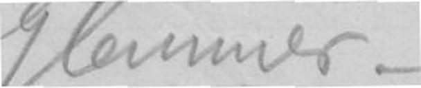glemmer
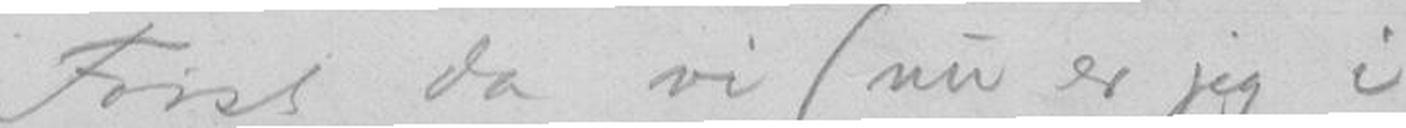Først da vi (nu er jeg i
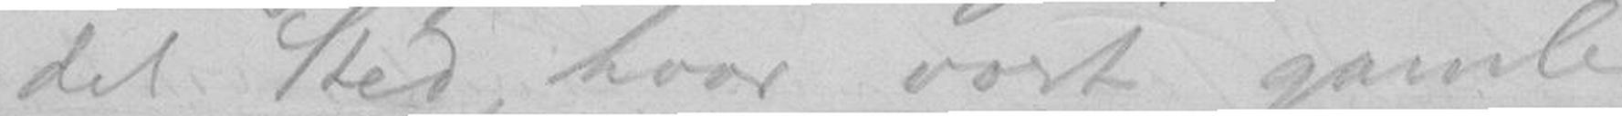det Sted hvor vort gamle
